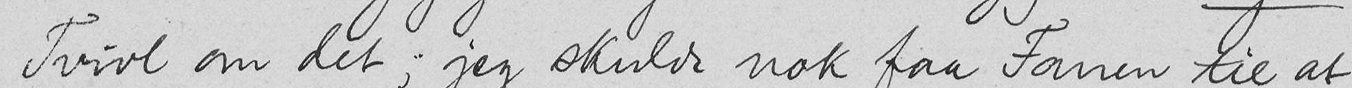Tvivl om det; jeg skulde nok faa Fanen til at
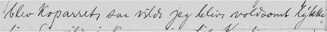blev koparret, saa vilde jeg blive voldsomt lykke-
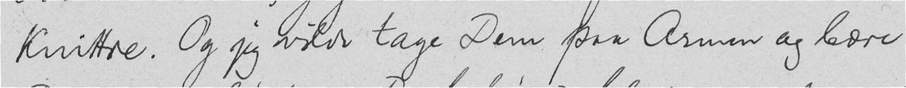knittre. Og jeg vilde tage Dem paa Armen og bære
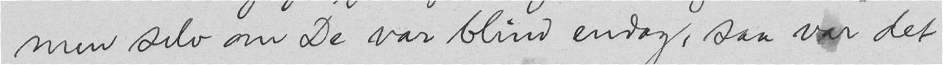men selv om De var blind endog, saa var det
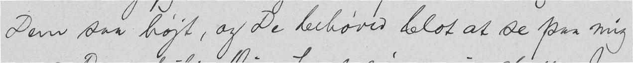Dem saa højt, og De behøved blot at se paa mig
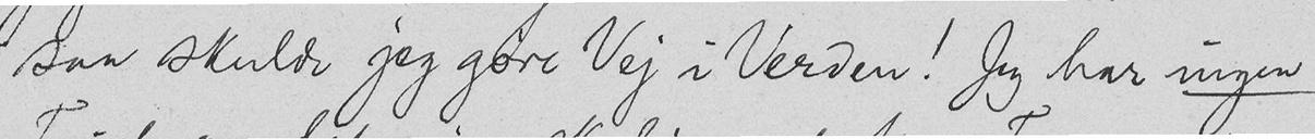saa skulde jeg gøre Vej i Verden! Jeg har ingen
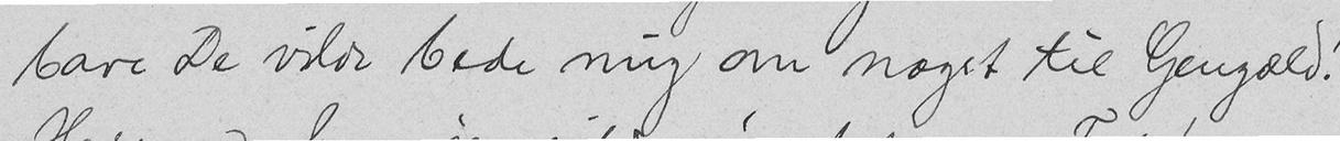bare De vilde bede mig om noget til Gengæld!
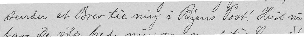sender et Brev til mig i Byens Post. Hvis nu
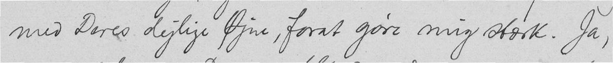med Deres dejlige Øjne, forat gøre mig stærk. Ja,
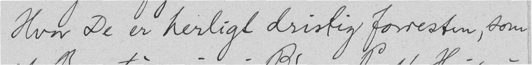Hvor De er herligt dristig forresten, som
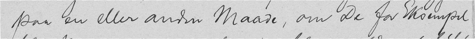paa en eller anden Maade, om De for Eksempel
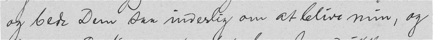og bede Dem saa inderlig om at blive min, og
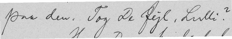paa den. Tog De fejl, Lulli?
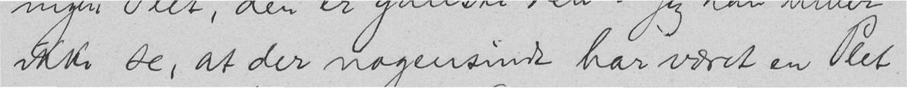ikke se, at der nogensinde har været en Plet
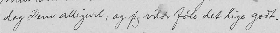dog Dem alligevel, og jeg vilde føle det lige godt.
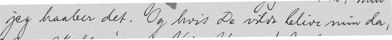jeg haaber det. Og hvis De vilde blive min da,
